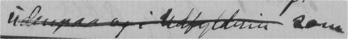som
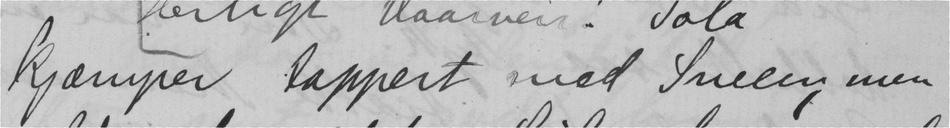Kjæmper tappert med Sneen; men
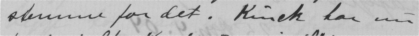stemme for det. Kinck har nu
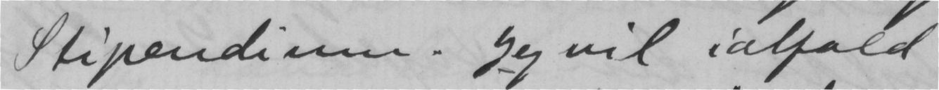Stipendium. Jeg vil ialfald
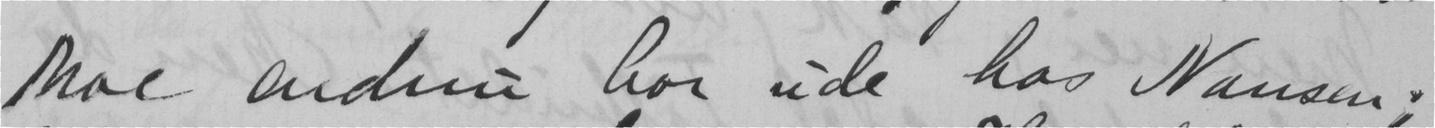Moe endnu bor ude hos Nansen;
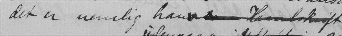det er nemlig han
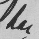har
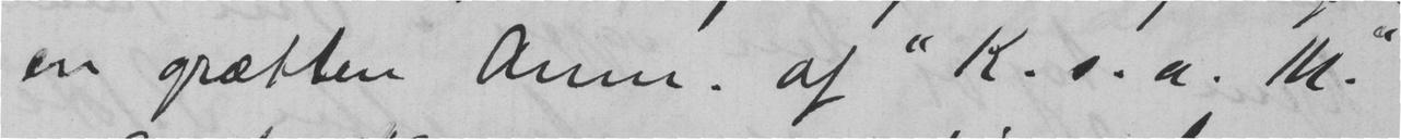en grætten Anm. af "K.s.a.M."
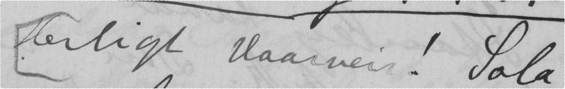Herligt Vaarveir! Sola
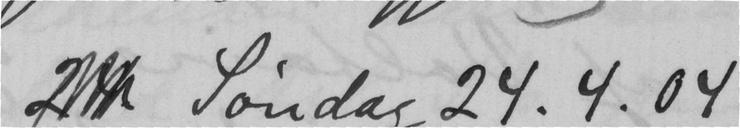Søndag 24.4.04
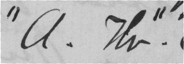"A. Hv".
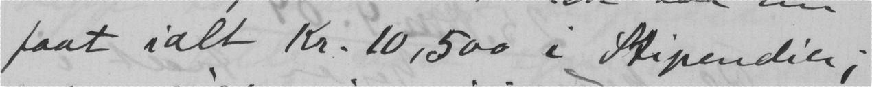faat ialt Kr. 10,500 i Stipendier
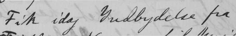Fik idag Indbydelse fra
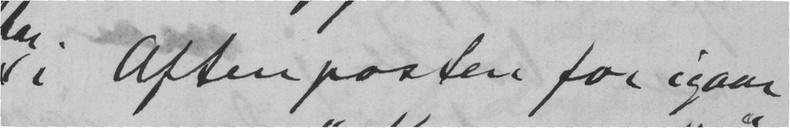i Aftenposten for igaar
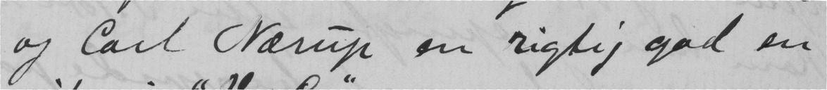og Carl Nærup en rigtig god en
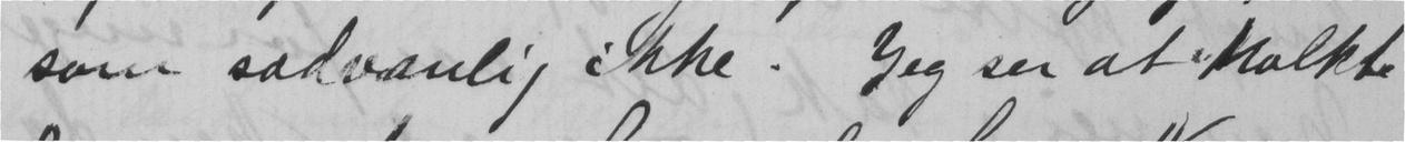som sædvanlig ikke. Jeg ser at Molkte
